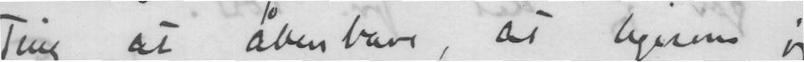Ting at åbenbare, at ligesom jeg
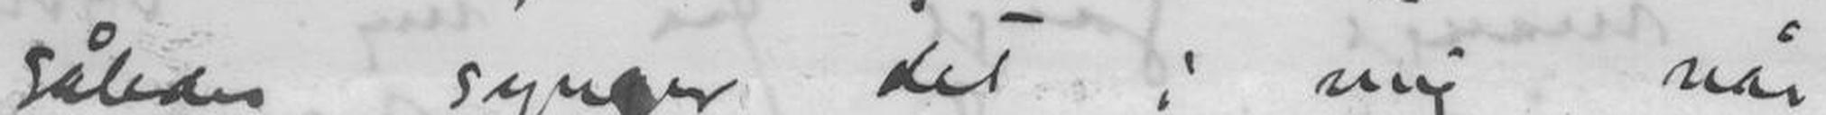således synger det i mig, når
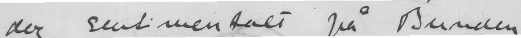dog sentimentalt på Bunden
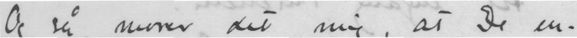Og så morer det mig, at De en-
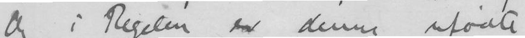Og i Regelen er denne ufødte
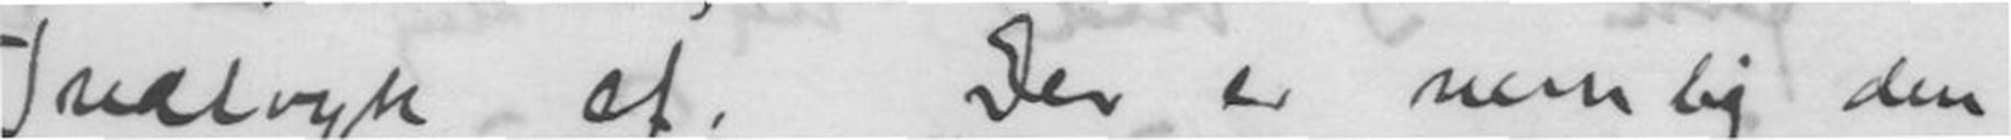Indtryk af. Der er nemlig den
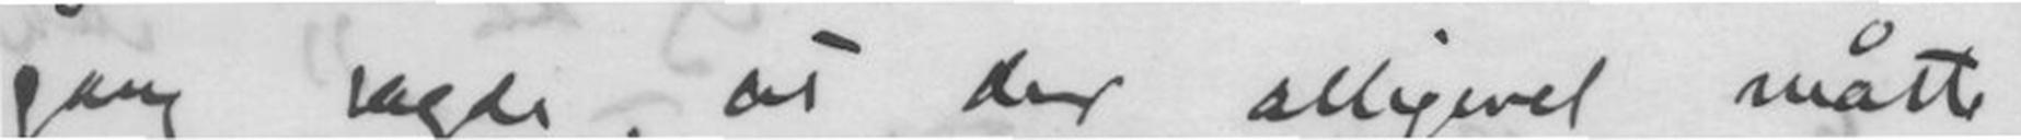gang sagde, at der alligevel måtte
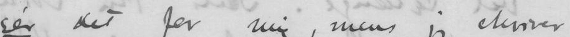ser det for mig, mens jeg skriver,
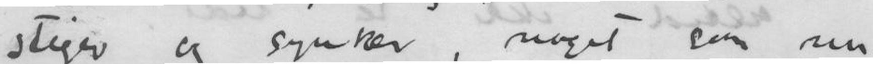stiger og synker, noget som nu
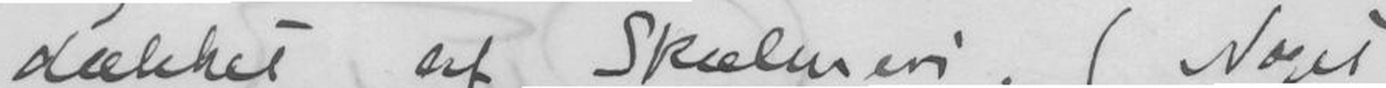dækket af Skæbneri. (Noget
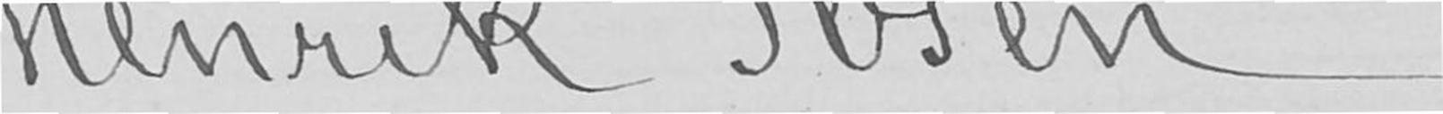Henrik Ibsen.
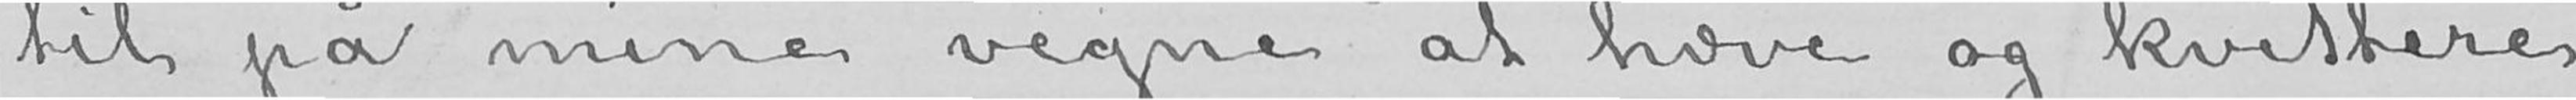til på mine vegne at hæve og kvittere
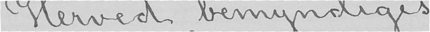Herved bemyndiges
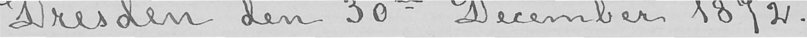Dresden den 30te December 1872.
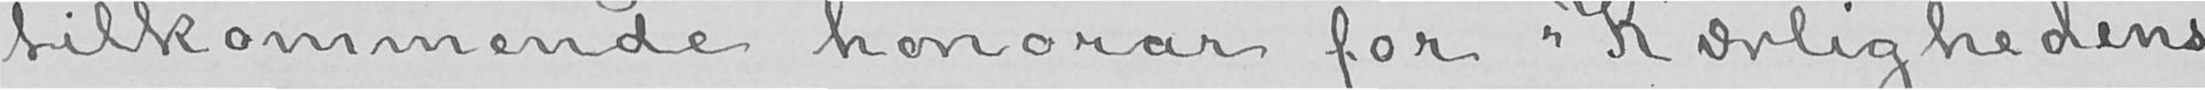tilkommende honorar for «Kærlighedens
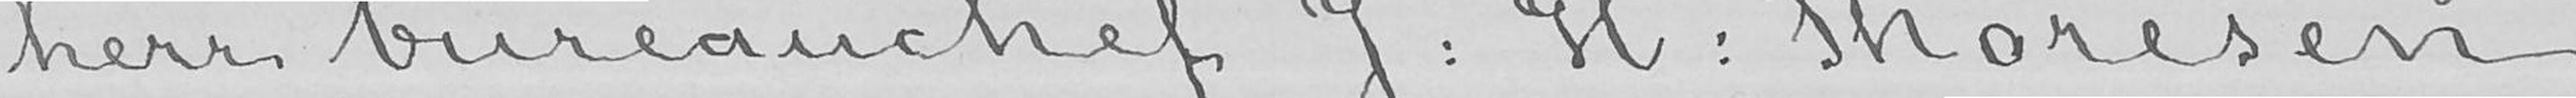herr bureauchef J: H: Thoresen
